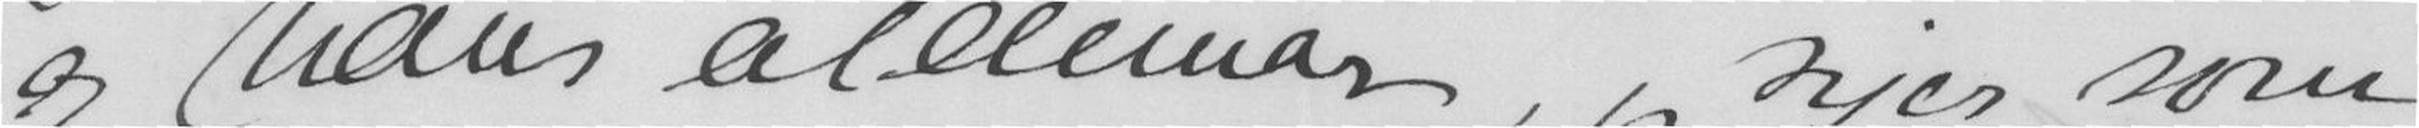og hans oldemor, jeg siger som
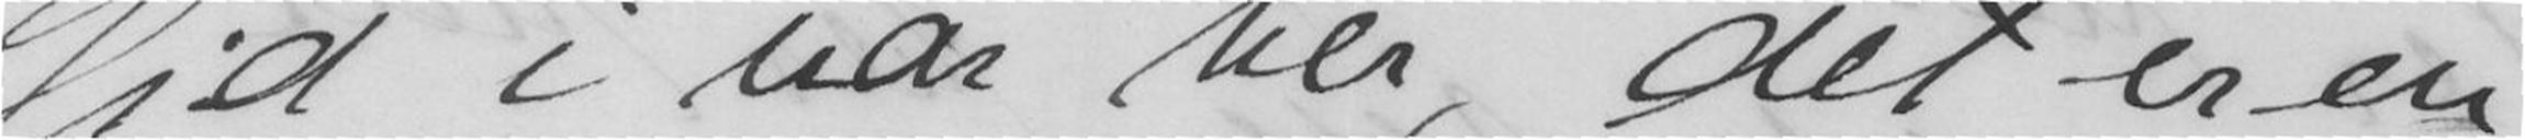Gid i var her, det er en
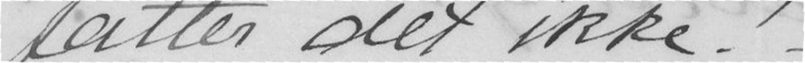fatter slet ikke!
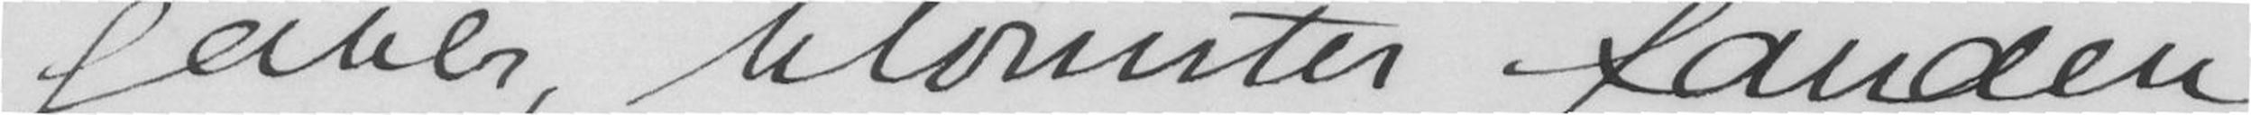Gaver, blomster fanden
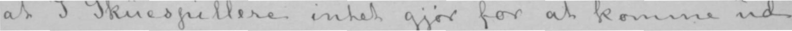at I Skuespillere intet gjør for at komme ud
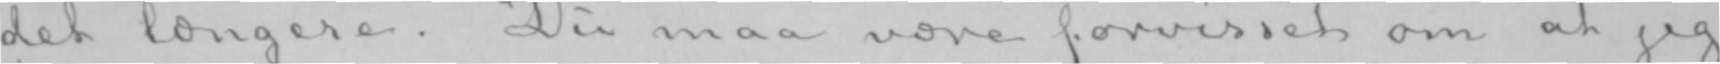det længere. Du maa være forvisset om at jeg
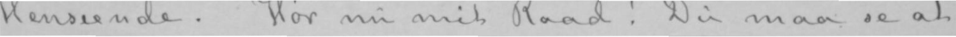Henseende. Hør nu mit Raad! Du maa se at
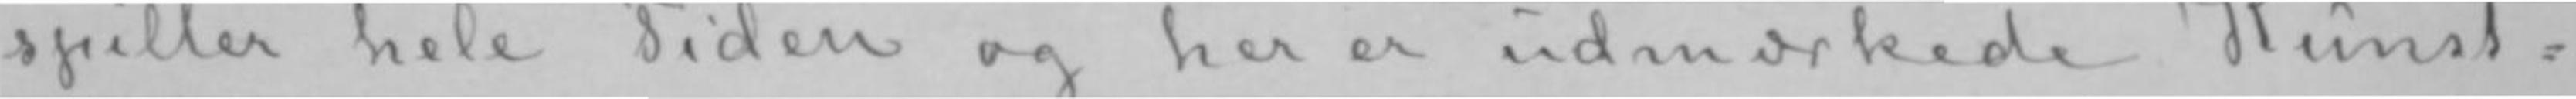spiller hele Tiden og her er udmærkede Kunst-
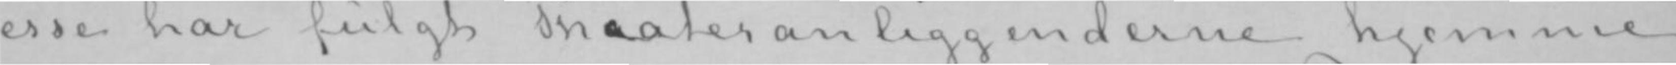esse har fulgt Theateranliggenderne hjemme
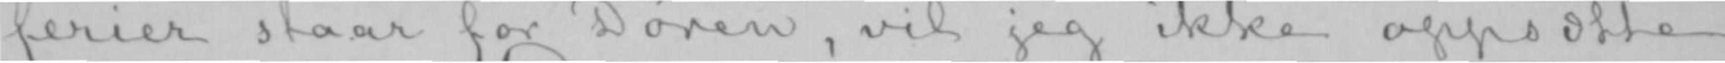ferier staar for Døren, vil jeg ikke oppsætte
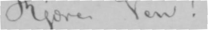Kjære Ven!
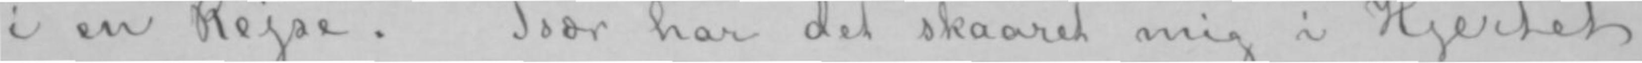i en Rejse. Især har det skaaret mig i Hjertet
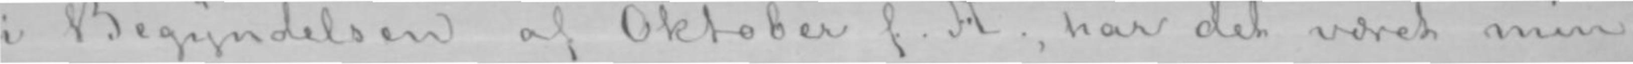i Begyndelsen af Oktober f. A., har det været min
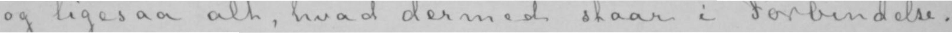og ligesaa alt, hvad dermed staar i Forbindelse.
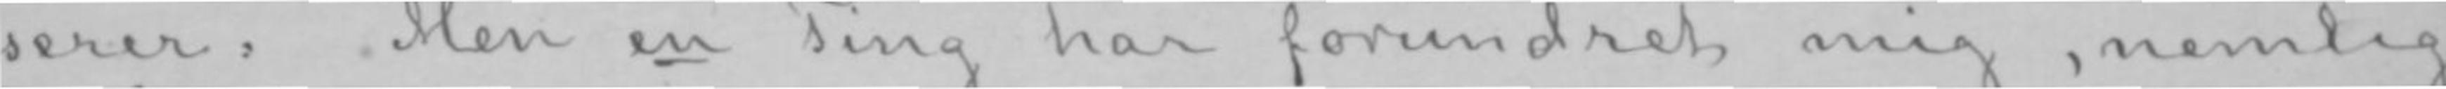serer. Men en Ting har forundret mig, nemlig
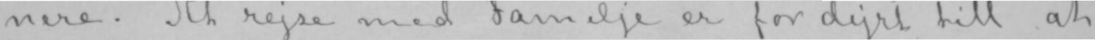nere. At rejse med Familje er for dyrt till at
